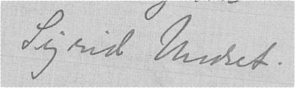Sigrid Undset.
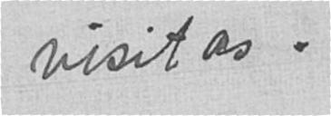visitas.
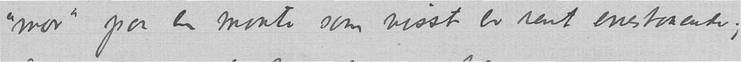"mor" paa en maate som visst er rent enestaaende;
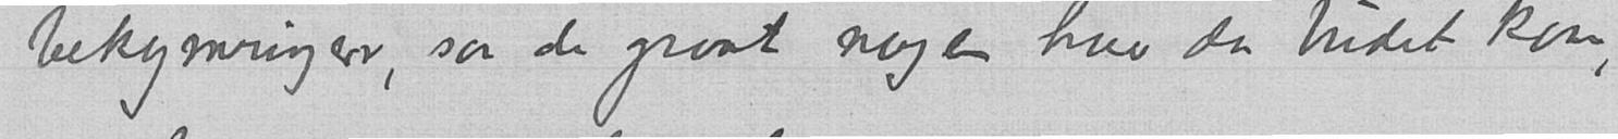bekymringer, saa de graat nogen hver da budet kom,
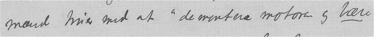mænd truer med at "demontere motoren og bære
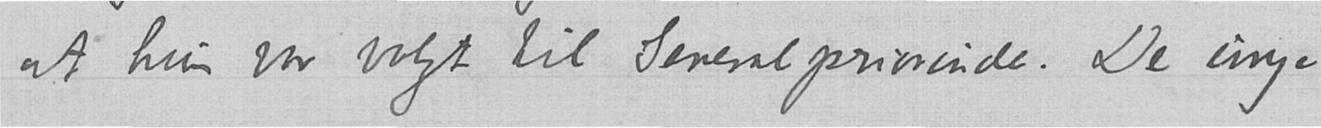at hun var valgt til Generalpriorinde. De unge
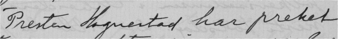Presten Hognestad har preket
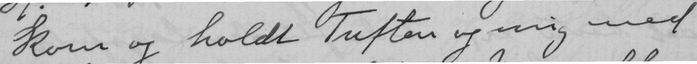kom og holdt Tuften og mig med
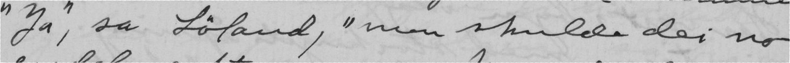"Ja", sa Løland, "men skulde dei no
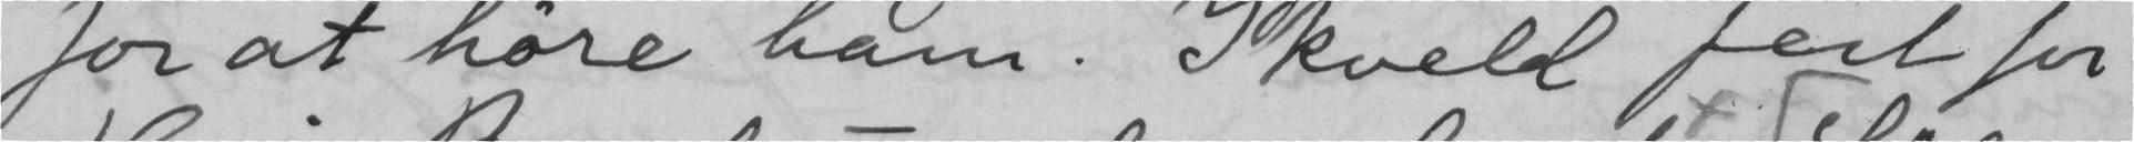for at høre ham. Ikveld fest for
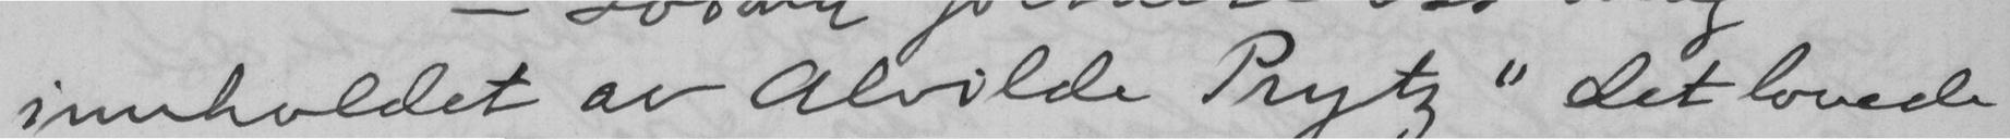innholdet av Alvilde Prytz "det lovede
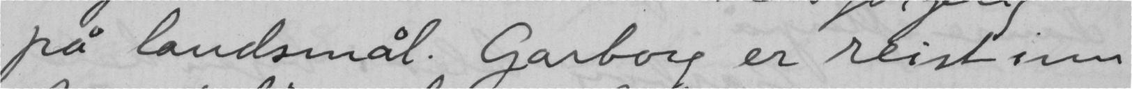på landsmål. Garborg er reist inn
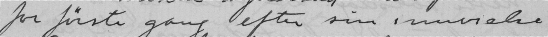for første gang efter sin innvielse
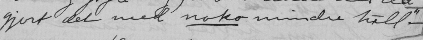gjort det med noko mindre tull." -
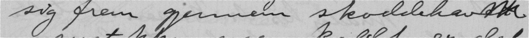sig frem gjennem skoddehav
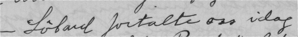- Løland fortalte oss idag
